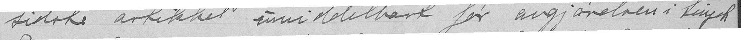sidste artikket umiddelbart før avgjørelsen i tinget.
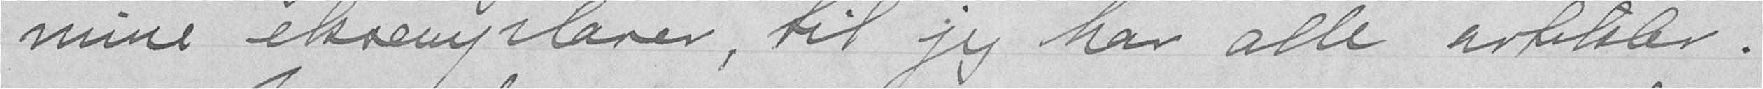mine eksemplarer, til jeg har alle artikler.
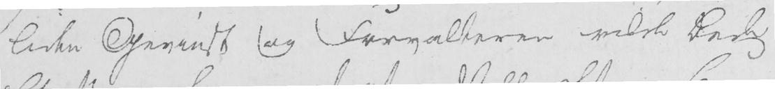liden Gevinst og Forvalteren vilde bede
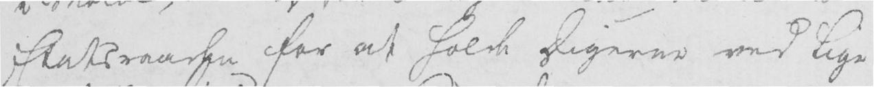Etatsraaden for at holde Digerne ved lige
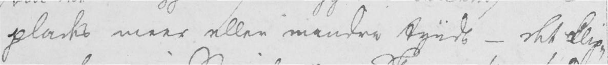plades meer eller mindee tyndt - det klip-
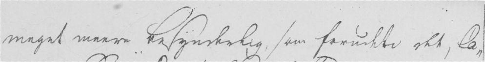meget meere besynderlig, som forudte det, Ca-
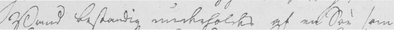Vand bestandig underholdes af en Søe som
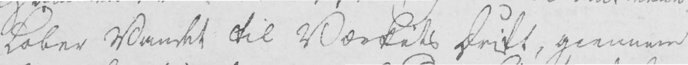løber Vandet til Værkets Drift, giennem
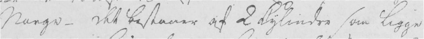Norge - det bestaaer af 2 Cylindre som ligge
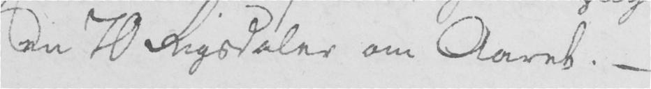en 70 Rigsdaler om Aaret. -
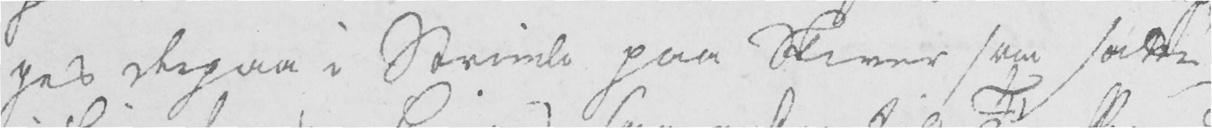pes derpaa i Strimle paa Skiver som sætte
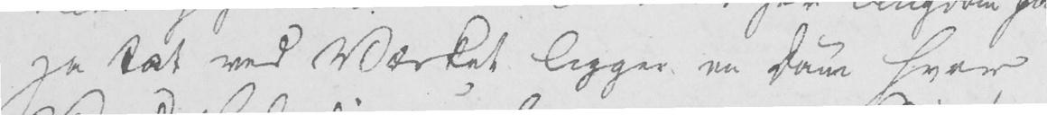ja tæt ved Værket ligger en Dam hvor
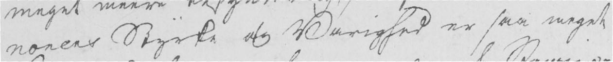nonens Styrke og Varighed er saa meget
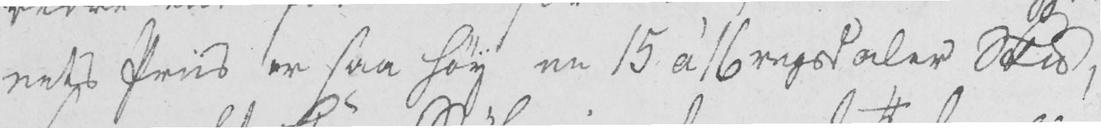nets Priis er saa høy en 15 à 16 rigsdaler Skd,
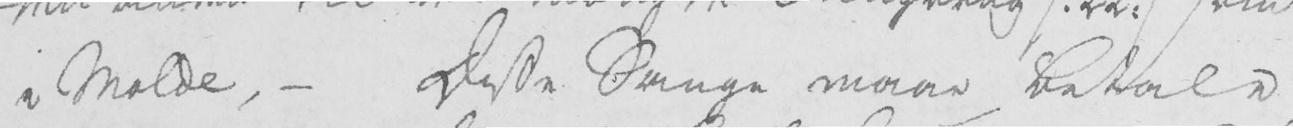i Molde, - disse Sauge maae betale
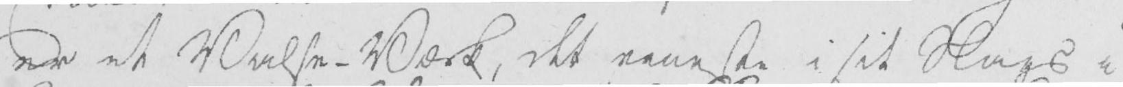er et Valse-Værk, det eeneste i sit Slags i
